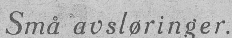Små avsløringer.
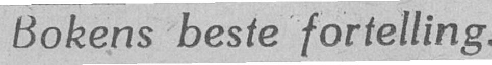Bokens beste fortelling.
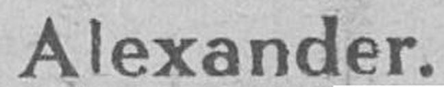Alexander.
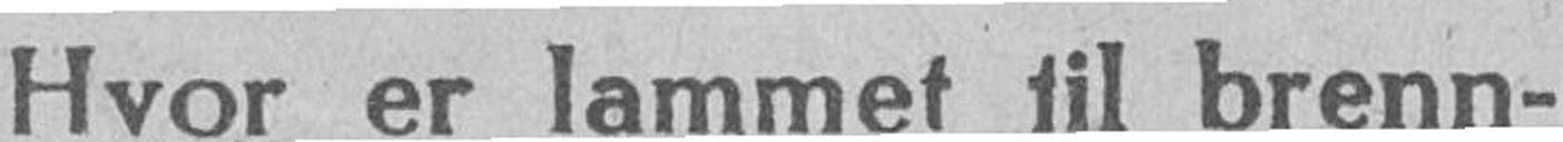Hvor er lammet fil brenn-
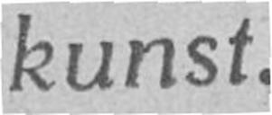kunst.
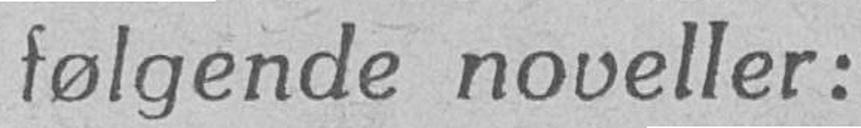følgende noveller:
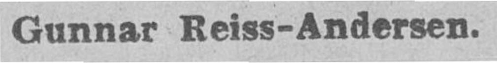Gunnar Reiss-Andersen.
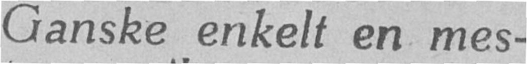Ganske enkelt en mes-
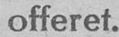offeret.
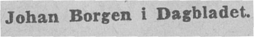Johan Borgen i Dagbladet.
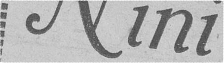Nini
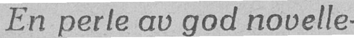En perle av god novelle-
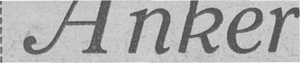Anker:
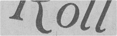Roll
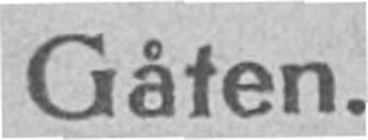Gåten.
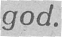god.
